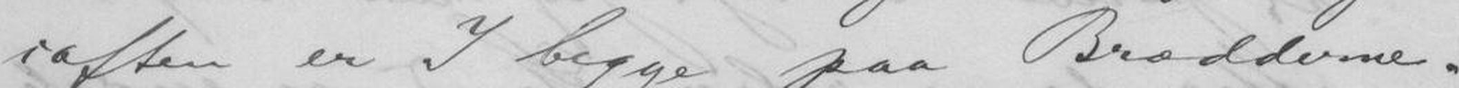iaften er I begge paa Brædderne.
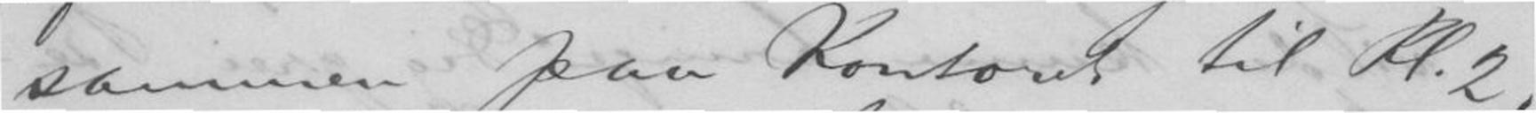sammen paa Kontoret til Kl.2,
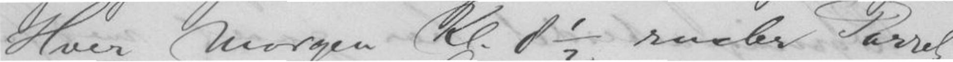Hver Morgen Kl. 8 ½ rusler Parret
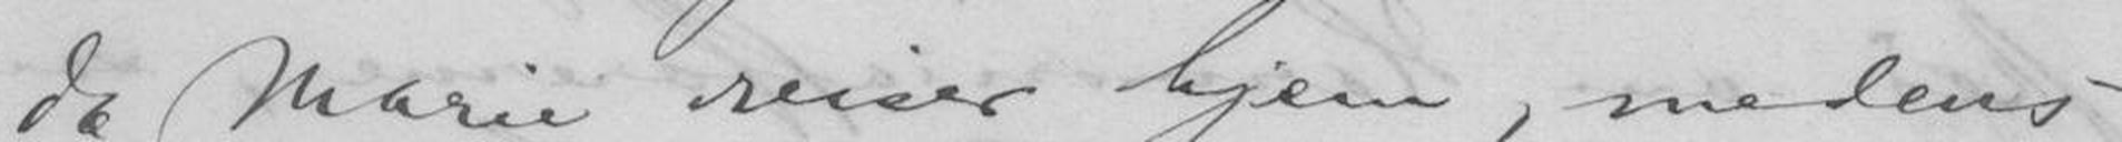da Marie reiser hjem, medens
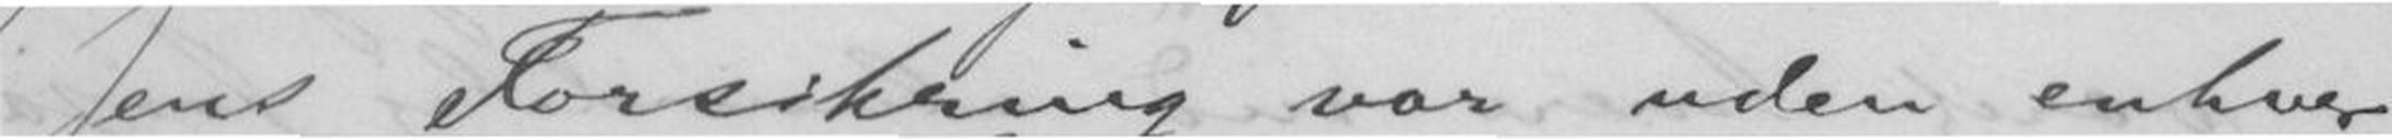sens Forsikring var uden enhver
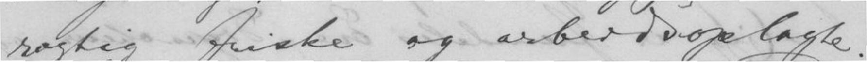rigtig friske og arbeidsoplagte.
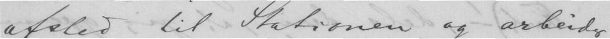afsted til Stationen og arbeider
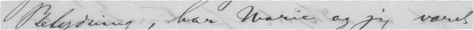Betydning, har Marie og jeg været
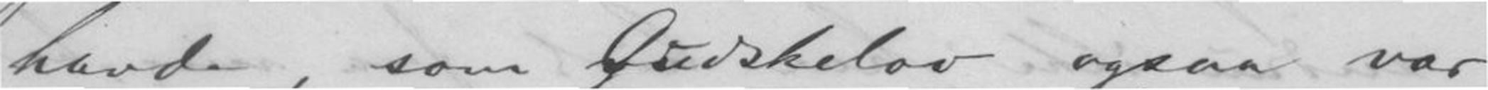havde, som Gudskelov ogsaa var
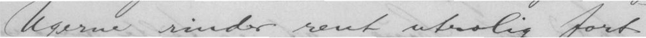Ugerne rinder rent utrolig fort
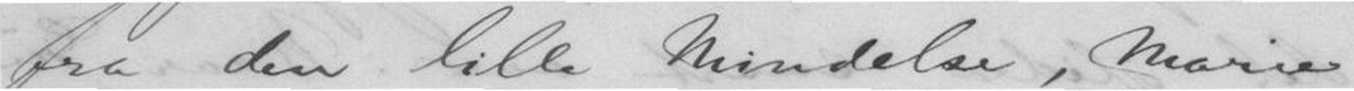fra den lille Mindelse, Marie
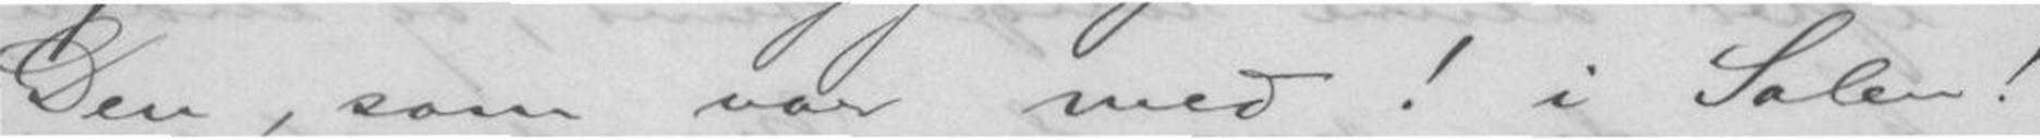Den, som var med! i Salen!
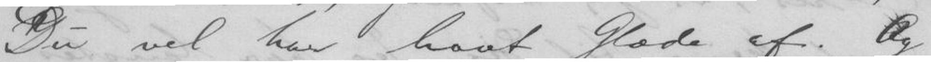Du vel har havt Glæde af. Og
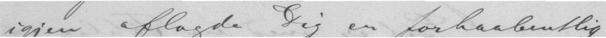igjen aflagde Dig en forhaabentlig
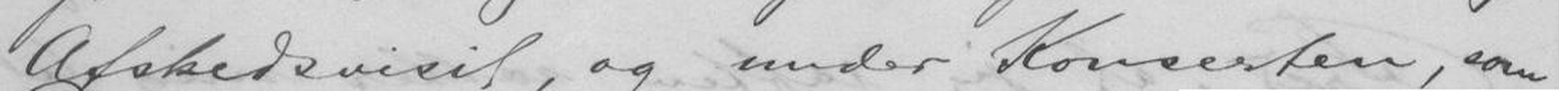Afskedsvisit, og under Konserten, som
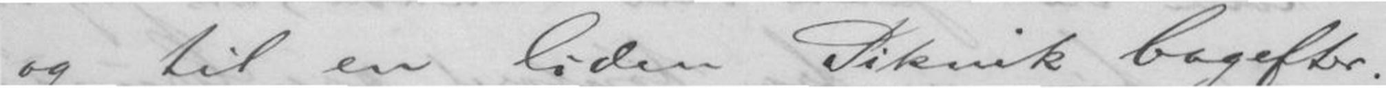og til en liden Piknik bagefter.
